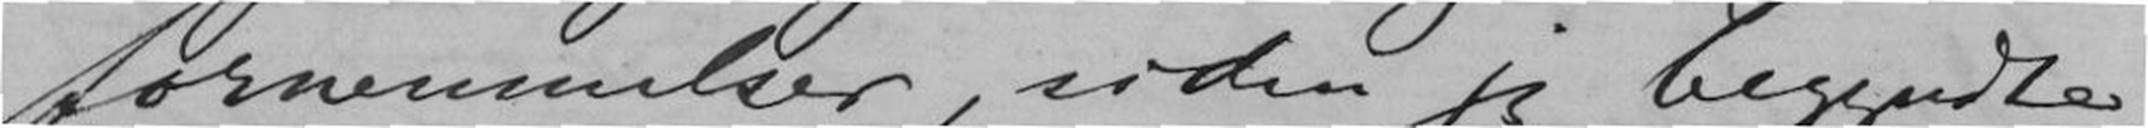fornemmelser, siden jeg begyndte
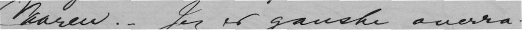Vaaren. - Jeg er ganske overra-
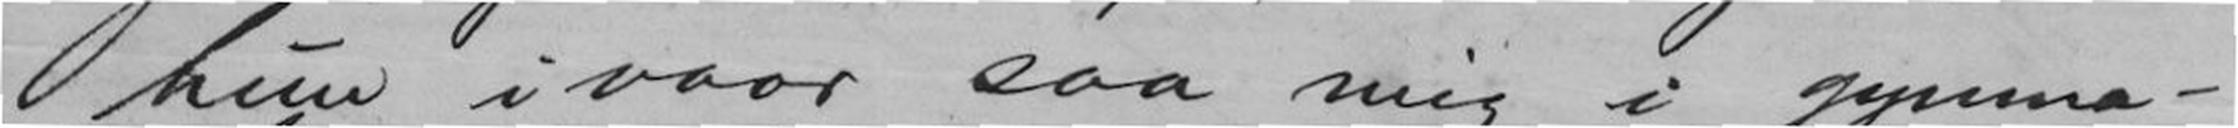hun ivaar saa mig i gymna-
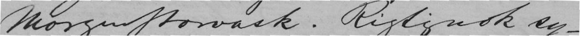Morgenstorvask. Rigtignok sy-
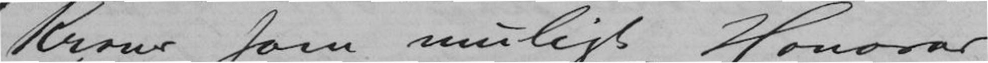Kroner som muligt Honorar
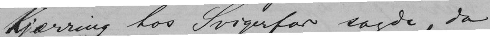Kjærring hos Svigerfar sagde, da
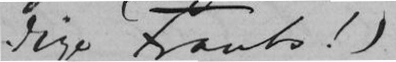dige Frants!))
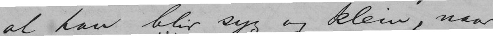at han blir strek under blir syg og klein, naar
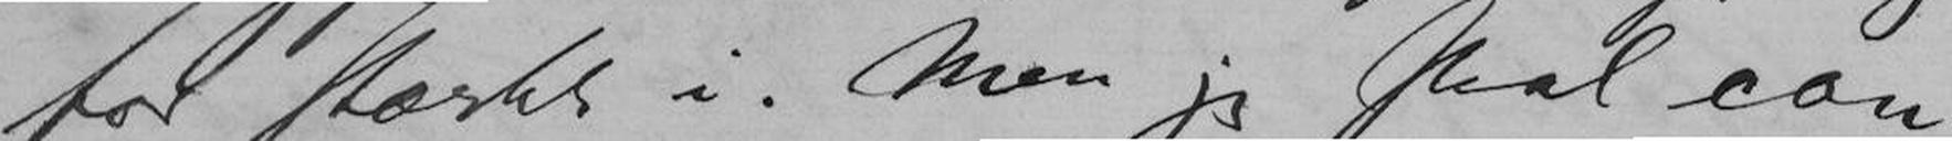for stærkt i. Men jeg skal con-
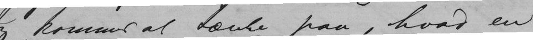Jeg kommer at tænke paa, hvad en
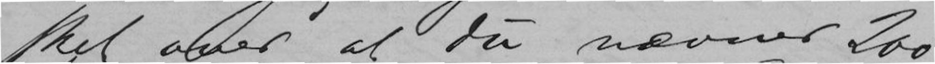sket over at du nævner 200
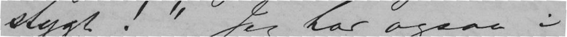stygt!" Jeg har ogsaa i
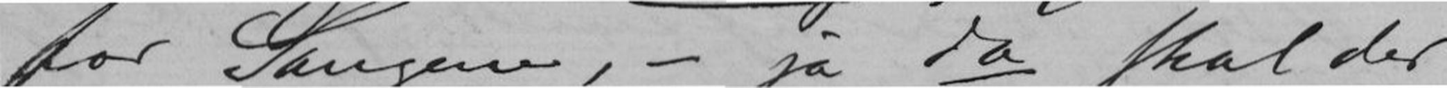for Sangene, - ja da skal der
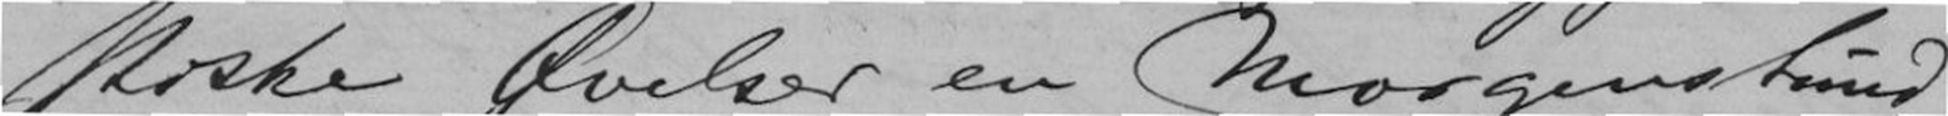stiske Øvelser en Morgenstund
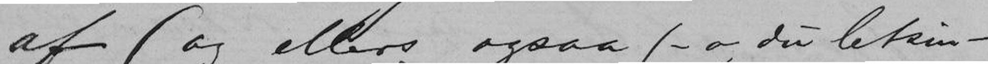af (og ellers ogsaa (- o, du letsin-
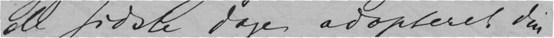de sidste dage adopteret din
